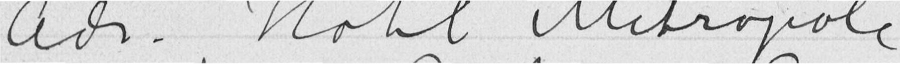Adr. Hotel Metropole
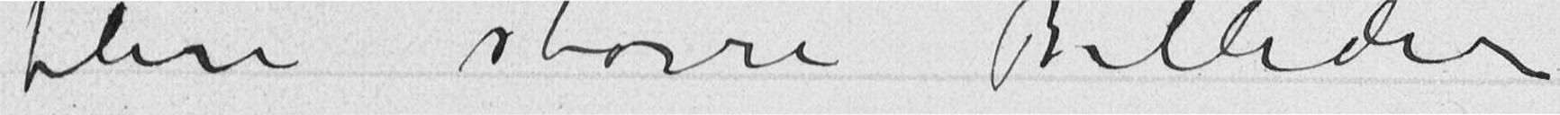flere større Billeder
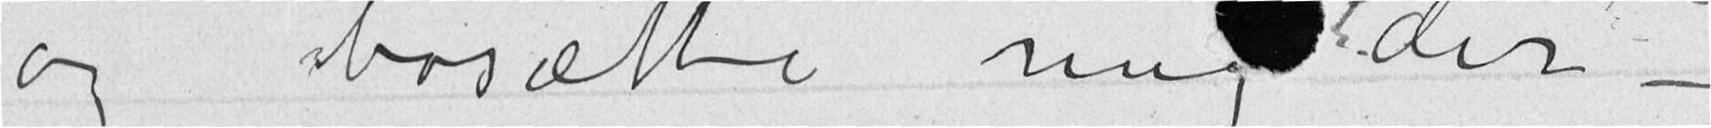og bosætte mig der –
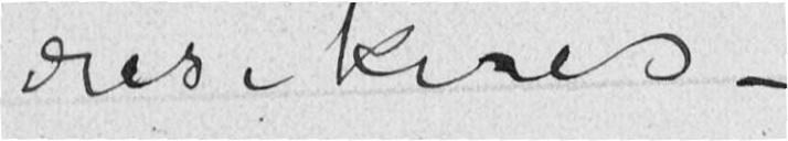risikeres –
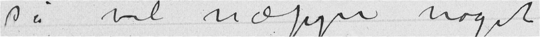så vil næppe noget
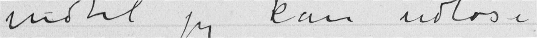indtil jeg kan udløse
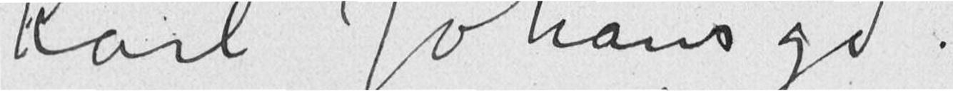Karl Johansgd.,
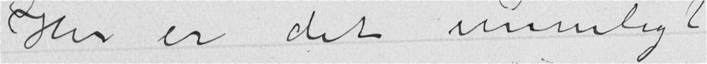Her er det umuligt
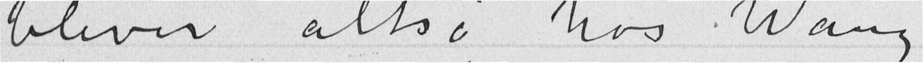bliver altså hos Wang
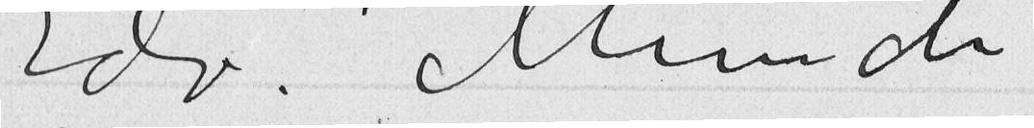Edv. Munch
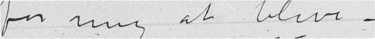for mig at blive –
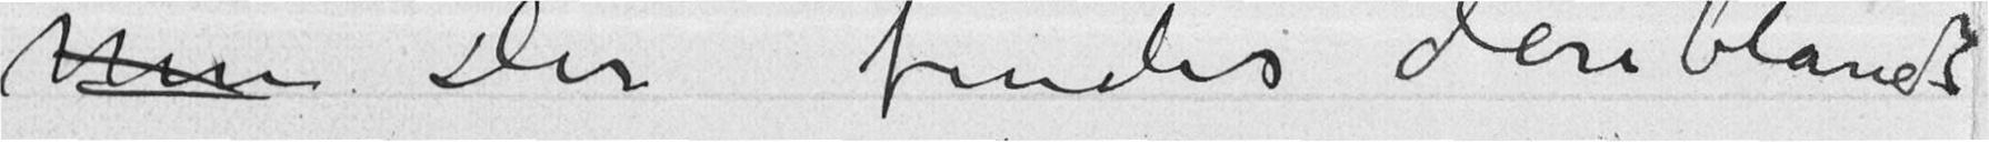Men Der findes deriblandt
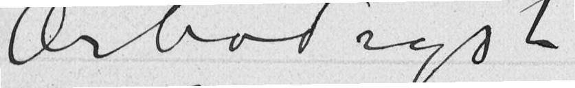Ærbødigst
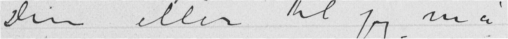dem eller til jeg må
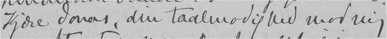Kjære Jonas, din taalmodighed mod mig
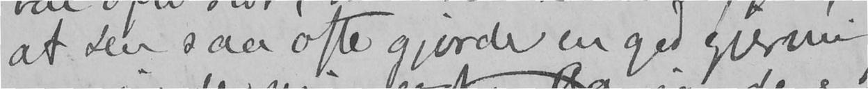at Du saa ofte gjorde en god gjerning,
